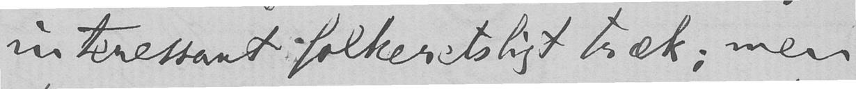interessant folkeretsligt træk; men
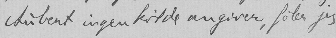Aubert ingen kilde angiver, føler jeg
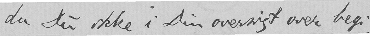da Du ikke i Din oversigt over begi-
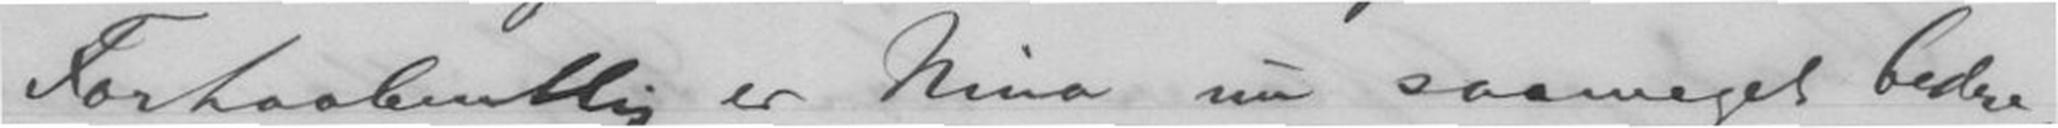Forhaabentlig er Nina nu saameget bedre,
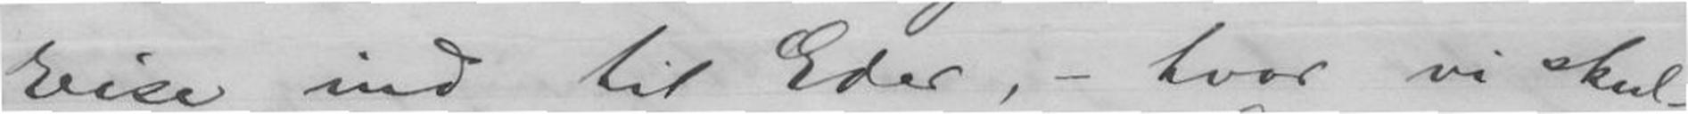reise ind til Eder, - hvor vi skul-
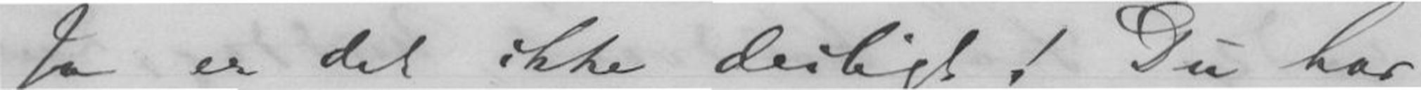Ja er det ikke deiligt! Du har
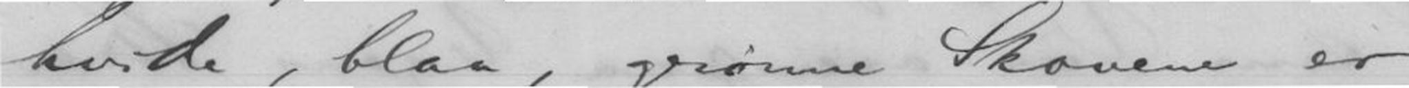hvide, blaa, grønne Skovene er
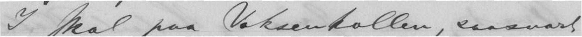I skal paa Voksenkollen, saasnart
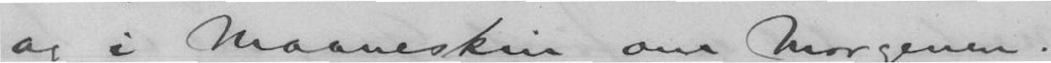og i Maaneskin om Morgenen.
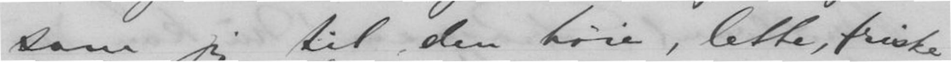som jeg til den høie, lette, friske
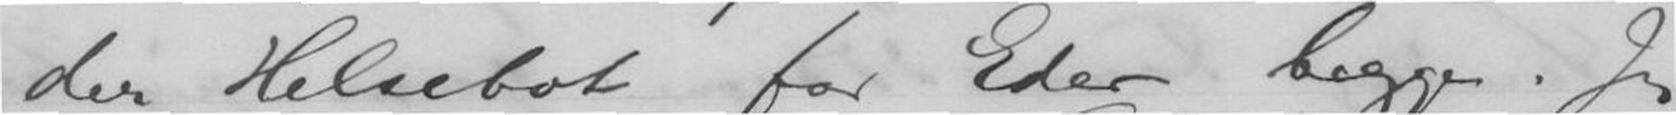der Helsebod for Eder begge. Jeg
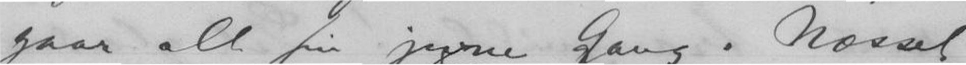gaar alt sin jevne Gang. Næsset
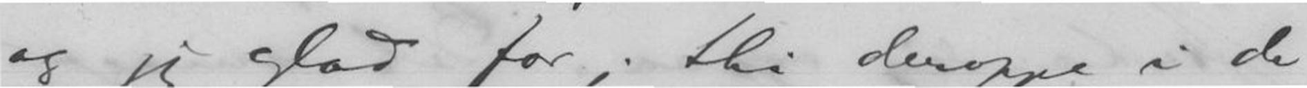og jeg glad for; thi deroppe i de
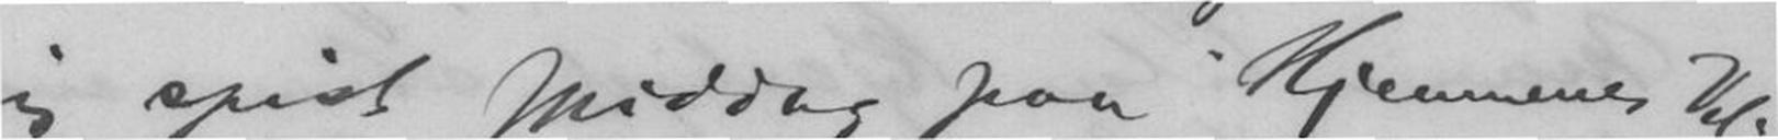jeg spist Middag paa "Hjemmenes Vel".
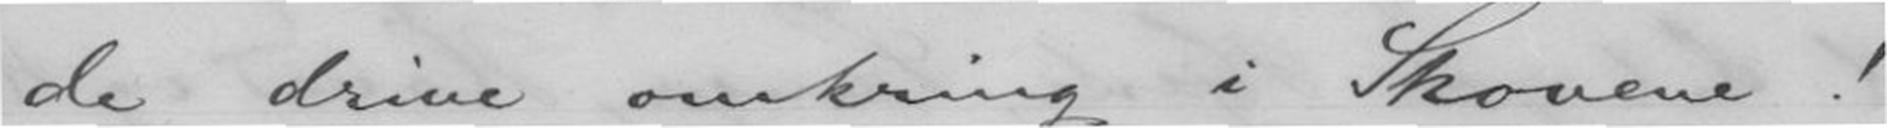de drive omkring i Skovene!
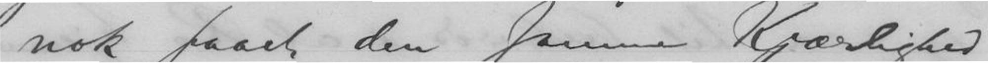nok faaet den samme Kjærlighed
